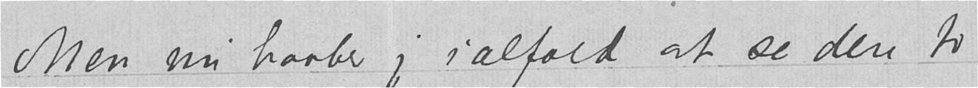Men nu haaber jeg ialfald at se dere to
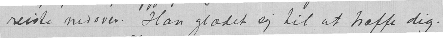reiste nedover. Han glædet sig til at træffe dig.
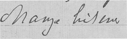Mange hilsener
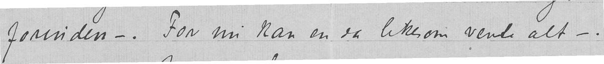forinden -. For nu kan en da likesom vente alt –.
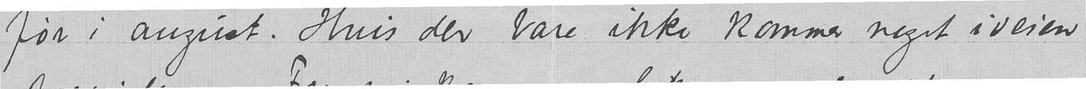før i august. Hvis der bare ikke kommer noget iveien
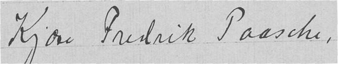Kjære Fredrik Paasche,
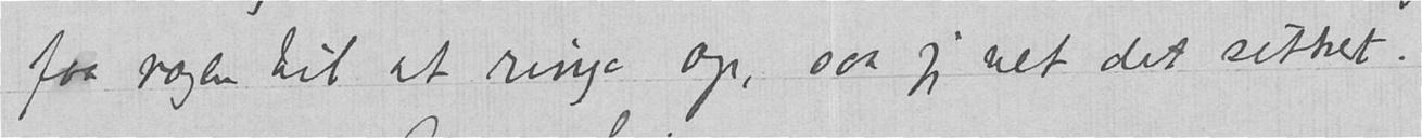faa nogen til at ringe op, saa jeg vet det sikkert.
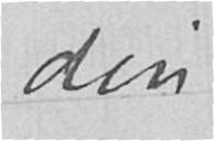din
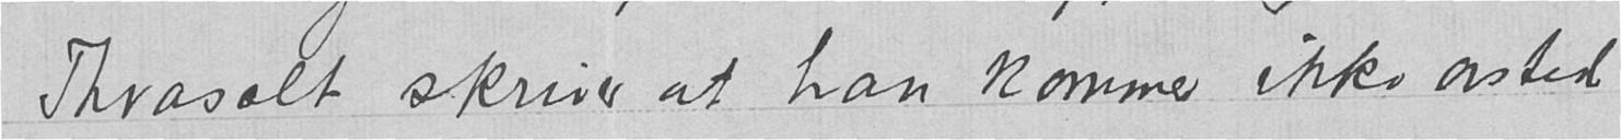Thrasolt skriver at han kommer ikke avsted
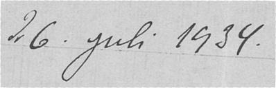26. juli 1934.
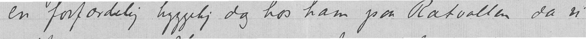en forfærdelig hyggelig dag hos ham paa Ratvollen da vi
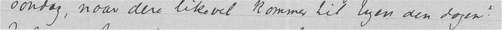søndag, naar dere likevel kommer til byen den dagen? -
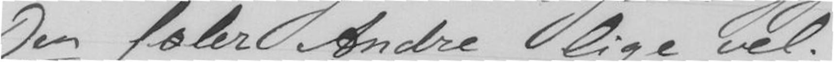Den føler Andre lige vel.
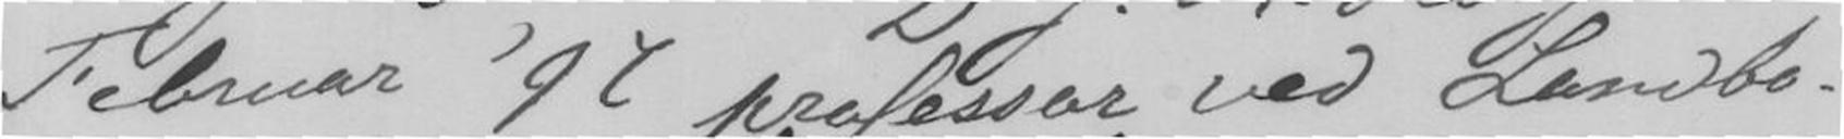Februar '97professor ved Landbo-
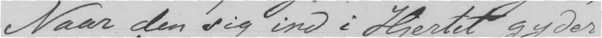Naar den sig ind i Hjertet gyder,
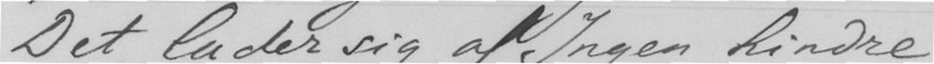Det lader sig af Ingen hindre
